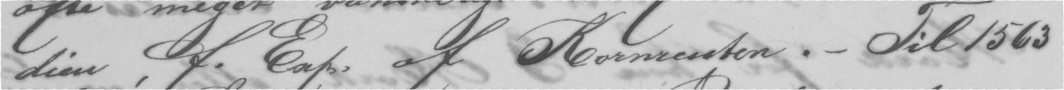dien, f. Exp, af Kornrenten. - Til 1563
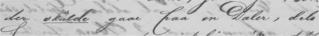der skulde gaae paa en Daler, dels
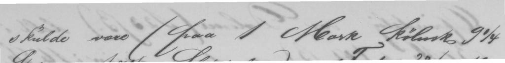skulde være (paa 1 Mark kølensk 9¼
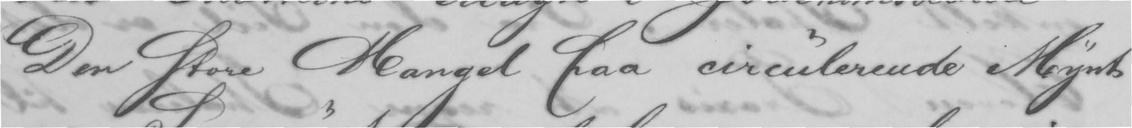Den Store Mangel paa circulerende Mynt
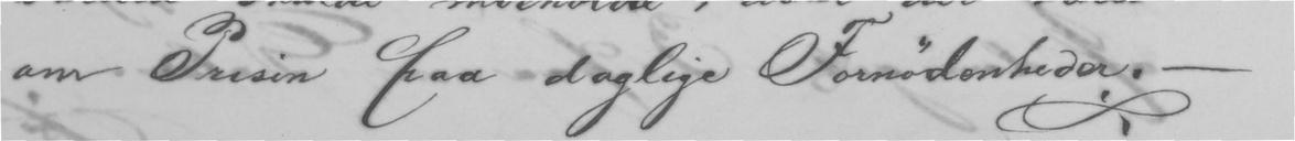om Presin paa daglige Fornødenheder. -
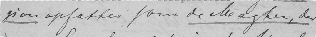gion opfattes som de Magter, der
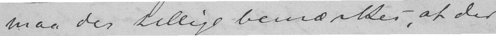maa det tillige bemærkes, at der
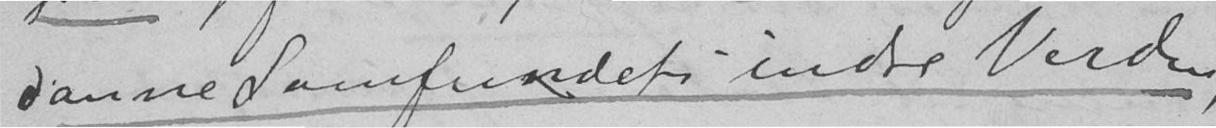danne Samfundets indre Verden,
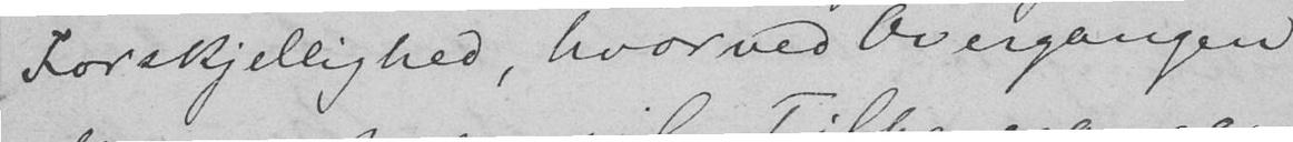Forskjellighed, hvorved Overgangen
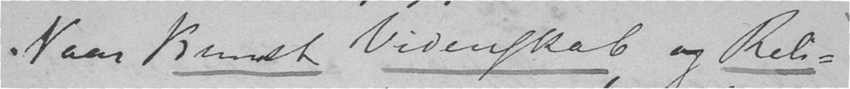Naar Kunst Videnskab og Reli-
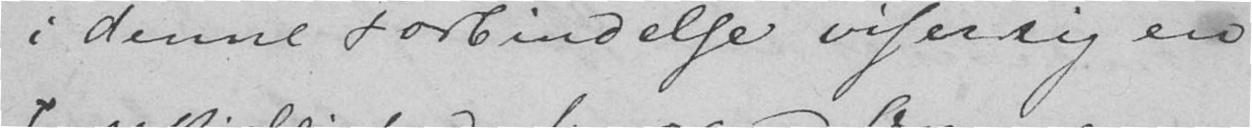i denne Forbindelse viser sig en
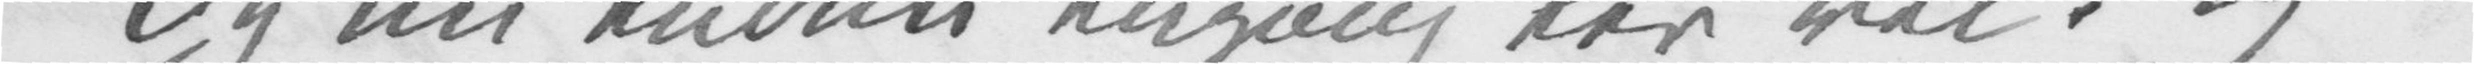Og nu endnu engang lev vel! og
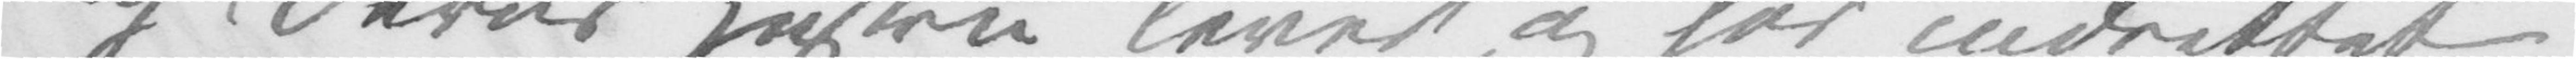og Deres Hustru lever og har indrettet
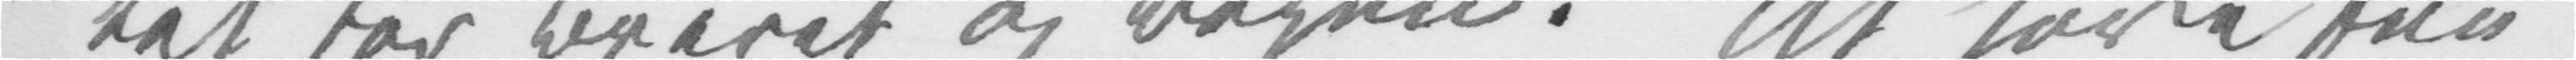tak for Brevet og Bogen. At høre fra
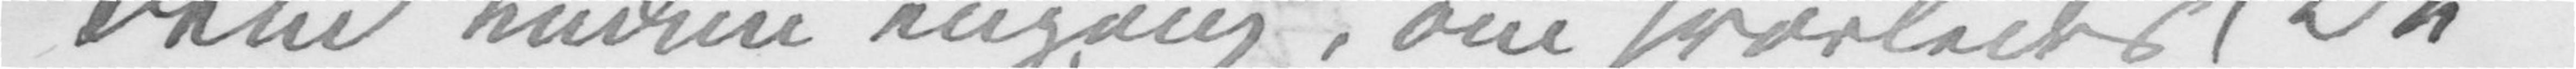Dem endnu engang, om hvorledes De
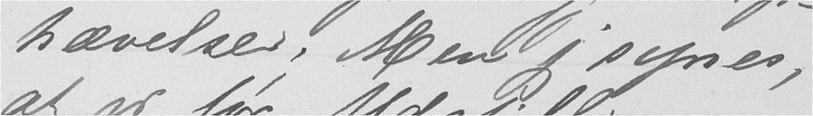hævelser. Men jeg synes,
